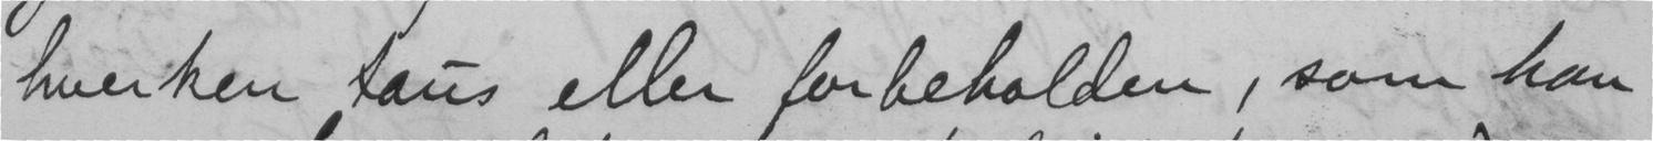hverken taus eller forbeholden, som han
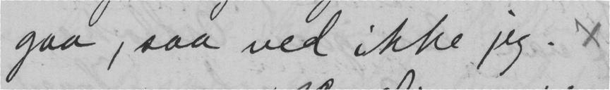gaa, saa ved ikke jeg. x
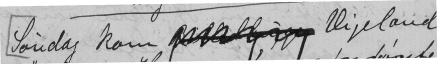Søndag kom  Vigeland
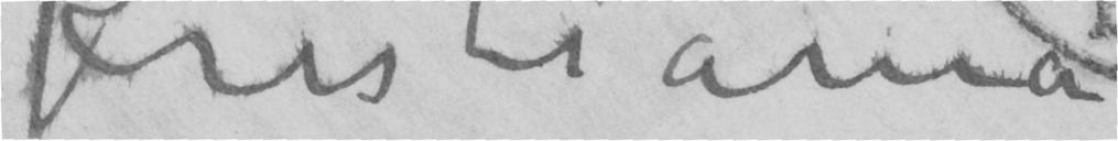Kristiania
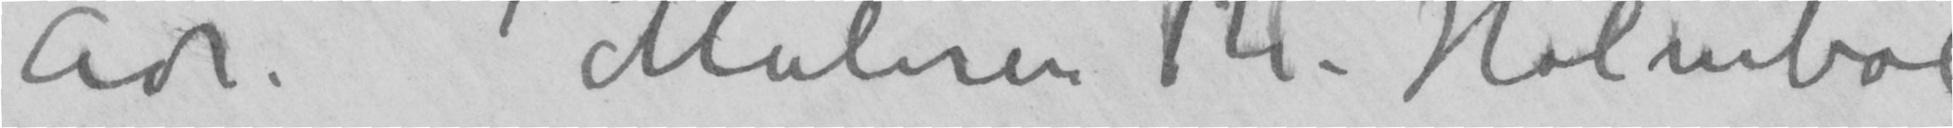Adr. Maleren Th. Holmboe
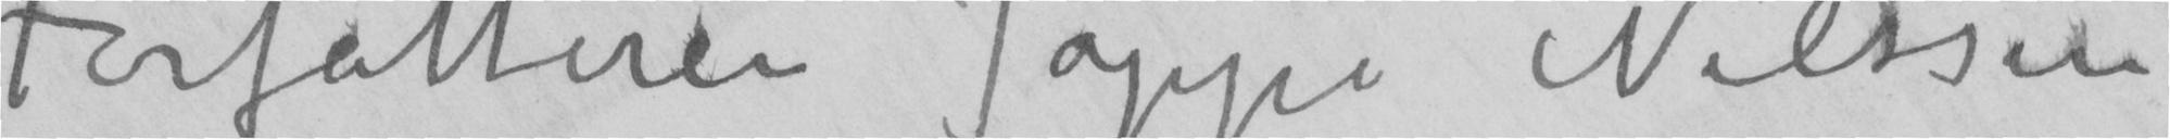Forfatteren Jappe Nilssen
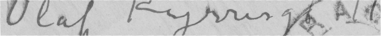S Olaf Kyrresgd 11
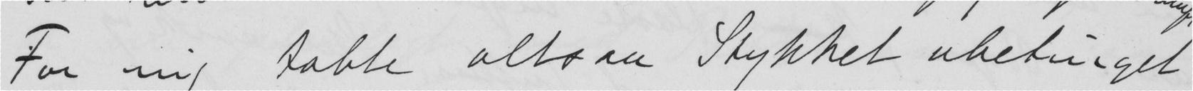For mig tabte altsaa Stykket ubetinget
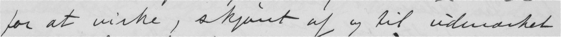for at virke, skjønt af og til udmærket
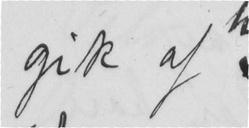gik af
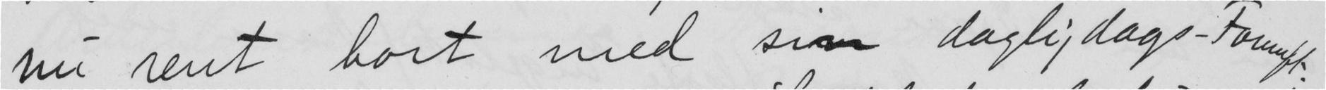nu rent bort med sin dagligdags-Fornuft.
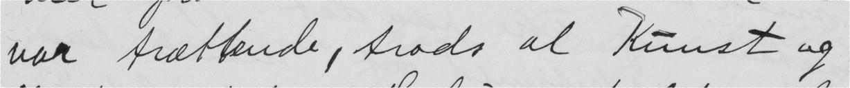var trættende, trods al Kunst og
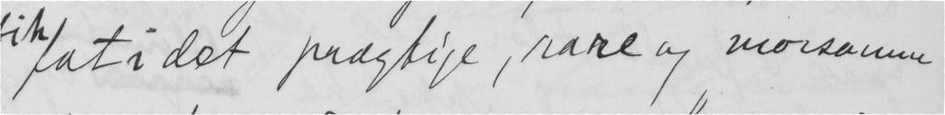fat i det prægtige, rare og morsomme
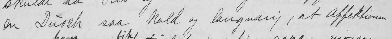en Dusch saa kold og langvarig, at Affektionen
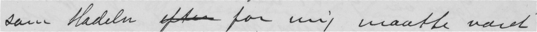som Hadeln  for mig maatte været
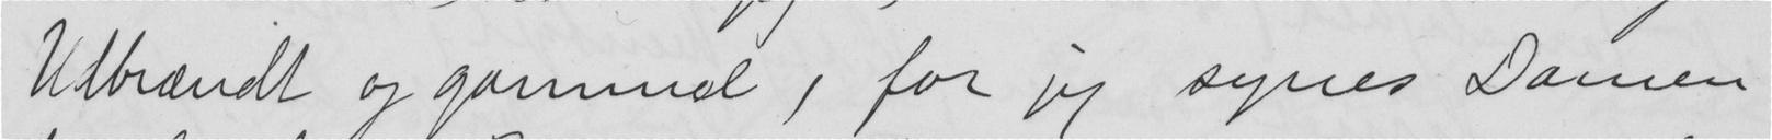Utbrændt og gammel, for jeg synes Damen,
